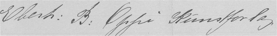Eberh. B. Oppi Kunstforlag
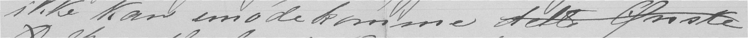ikke kan imødekomme dette Ønske
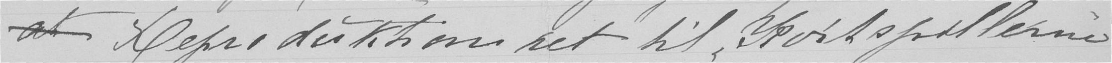at Reproduktionsret til "Kortspillerne
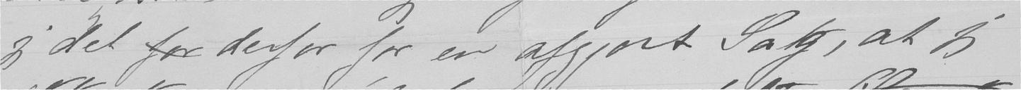jeg det for derfor for en afgjort Sag, at jeg
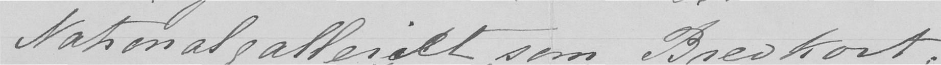Nationalgalleriet som Brevkort.
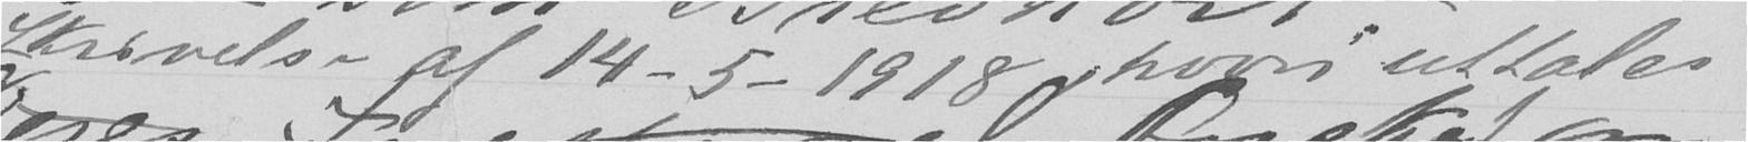Skrivelse af 14-5-1918, hvori uttales
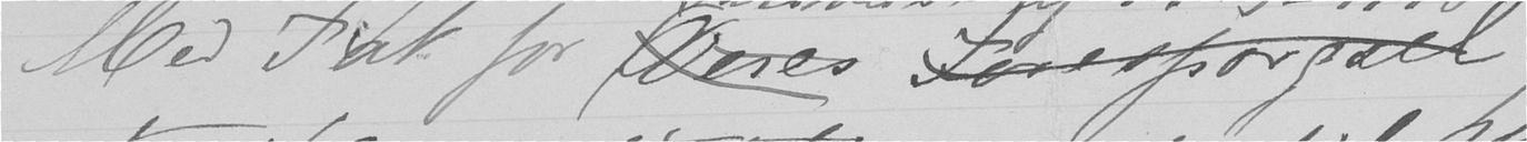Med Tak for Deres Forespørgsel
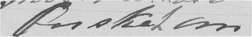Ønsket om
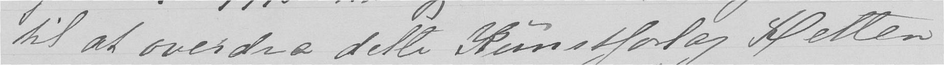til at overdra dette Kunstforlag Retten
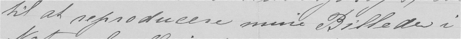til at reproducere mine Billeder i
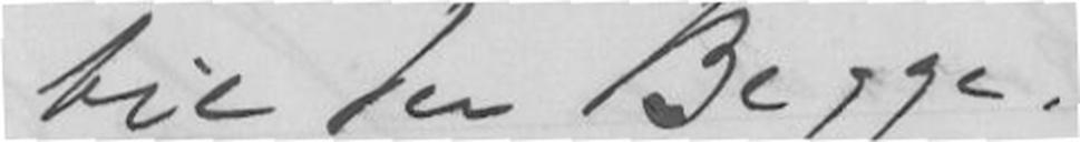til Jer Begge.
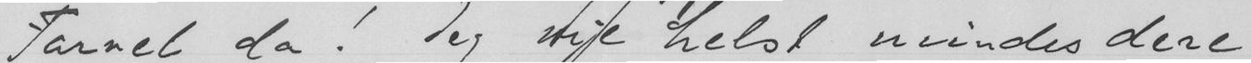Farvel da! Jeg vil helst mindes dere
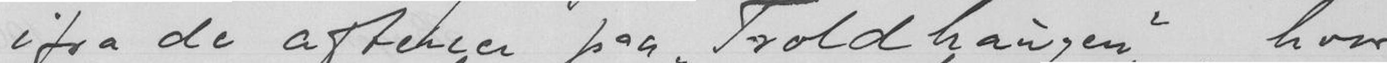ifra de aftener paa Troldhaugen, hvor
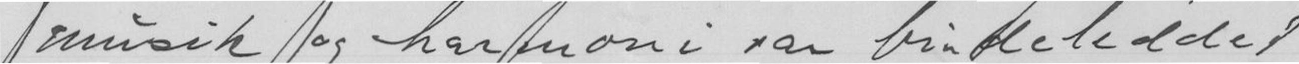musik og harmoni var bindeleddet
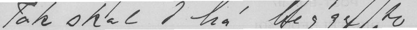Tak skal I ha' begge to!
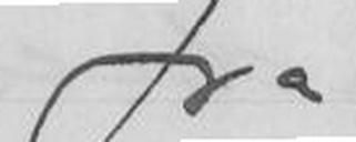fra
